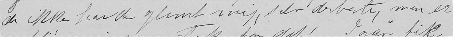de ikke havde glæmt mig, selv derborte, men er
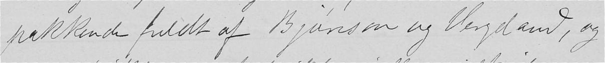pakkende fuldt af Bjørnson og Vergeland, og
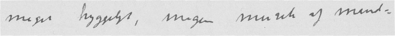meget hyggeligt, megen musik og mand-
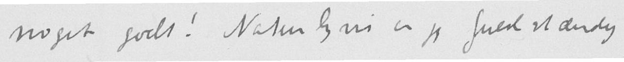noget godt! Naturligvis er jeg fuldstændig
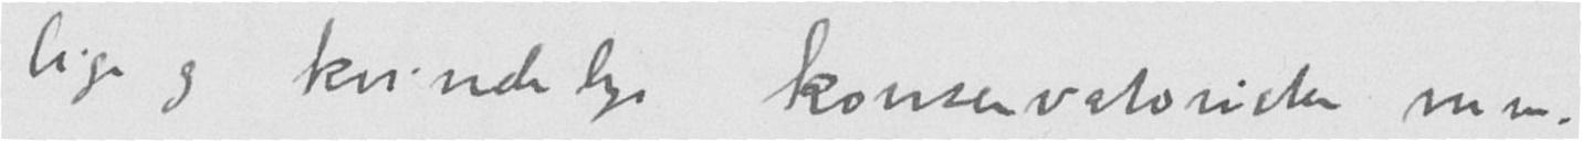lige og kvindelige konservatorister m m.
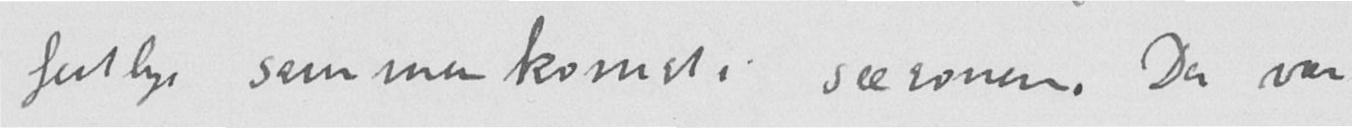festlige sammenkomst i sæsonen. Der var
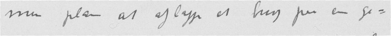min plan at aflægge et besøg paa en ge-
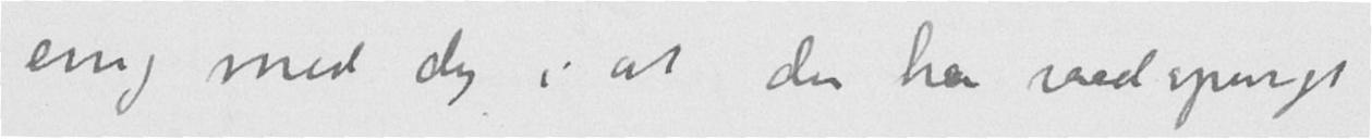enig med dig i at du har raadspurgt
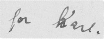for Karl.
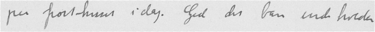paa posthuset i dag. Gid det bare indeholder
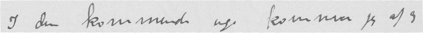I den kommende uge kommer jeg af og
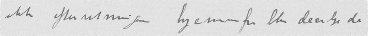ikke efterretninger hjemmfra blir daarlige da
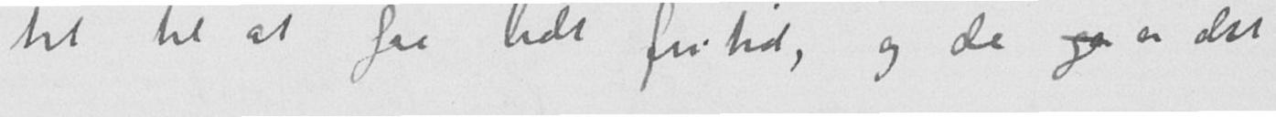til til at faa lidt fritid, og da ga er det
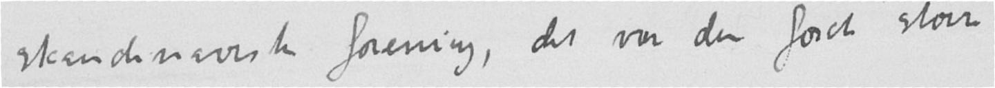skandinaviske forening, det var den første store
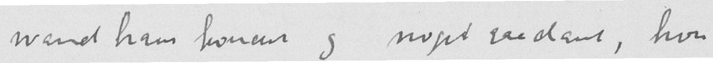wandhauskoncert og noget saadant, hvis
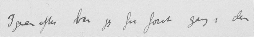I gaar aftes var jeg for første gang i den
